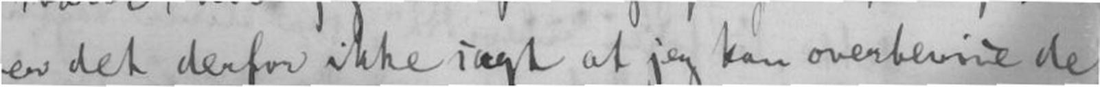er det derfor ikke sagt at jeg kan overbevise de
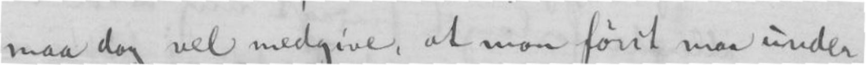maa dog vel medgive, at man først maa under-
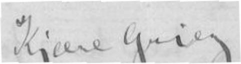Kjære Grieg!
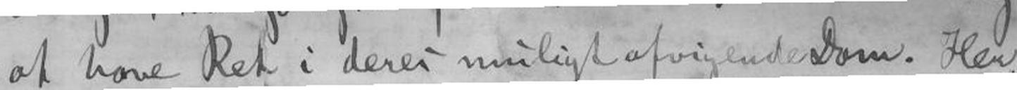at have Ret i deres muligt afvigende Dom. Her-
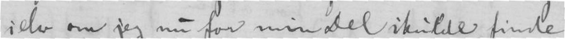selv om jeg nu for min Del skulde finde
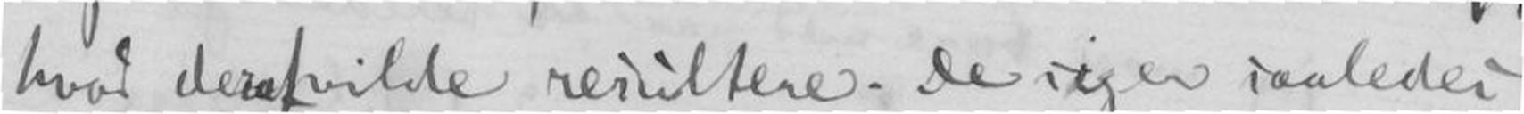hvad deraf vilde resultere. De siger saaledes
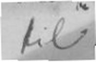til
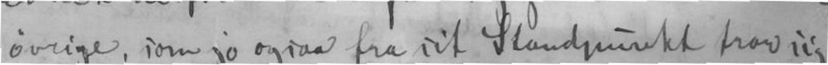øvrige, som jo ogsaa fra sit Standpunkt tror sig
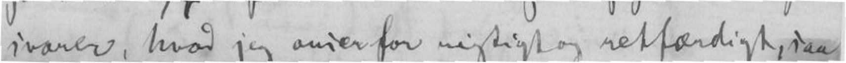svarer, hvad jeg anser for rigtigt og retfærdigt, saa
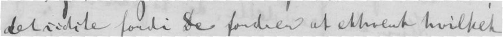det sidste fordi De fodrer at ethvert hvilket
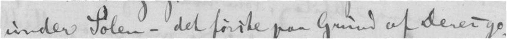under Solen - det første paa Grund af Deres go-
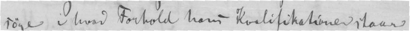søge i hvad Forhold hans Kvalifikationer staar
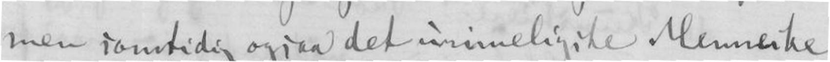men samtidig ogsaa det urimeligste Menneske
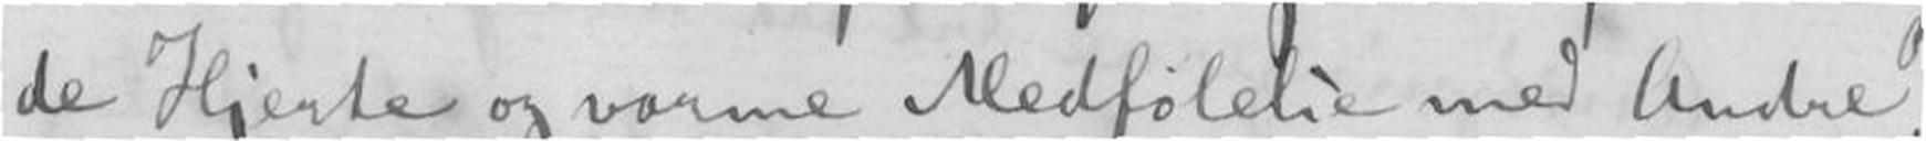de Hjerte og varme Medfølelser med Andre,
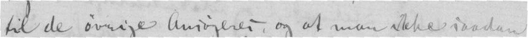til de øvrige Ansøgeres, og at man ikke saadan
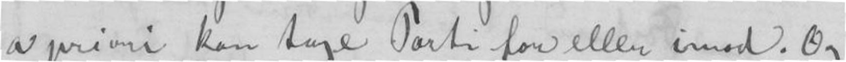a priori kan tage Parti for eller imod. Og
